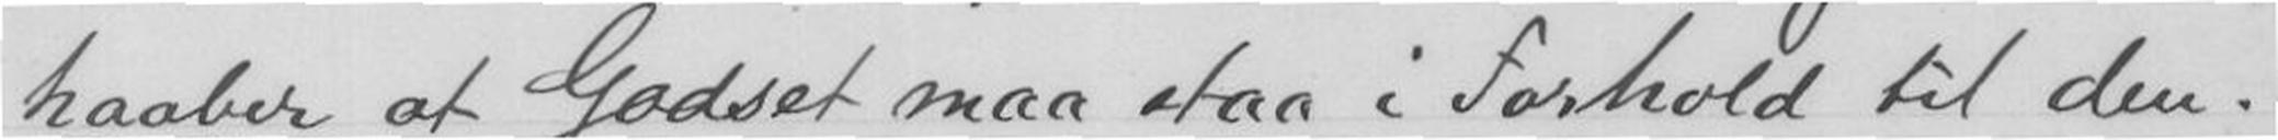haaber at Godset maa staa i Forhold til den.
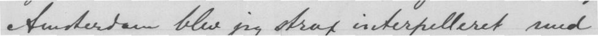Amsterdam blev jeg strax interpelleret med
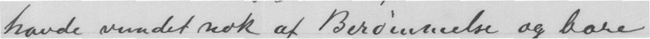havde vundet nok af Berømmelse og bare
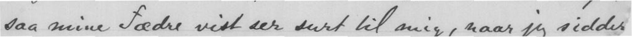saa mine Fædre vist ser surt til mig, naar jeg sidder
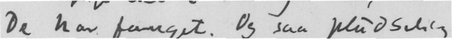De har fanget. Og saa pludselig
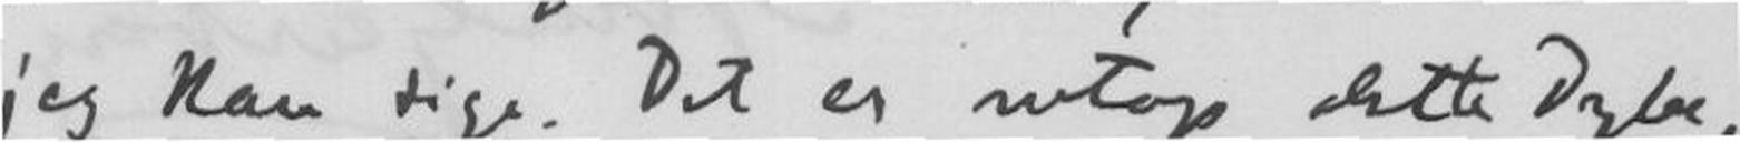jeg kan sige. Det er netop dette Dybe,
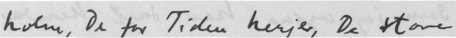holm, De for Tiden herje, De store
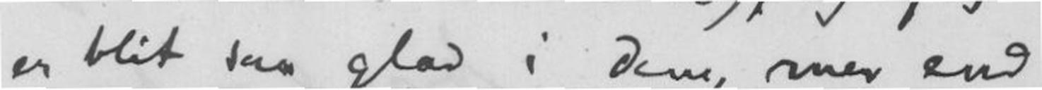er blit saa glad i dem, mer end
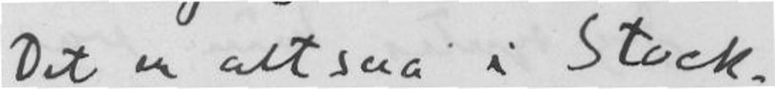Det er altsaa i Stock-
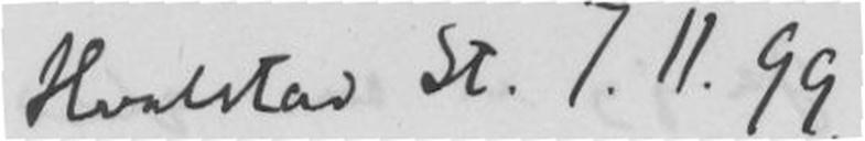Hvalstad St. 7.11.99.
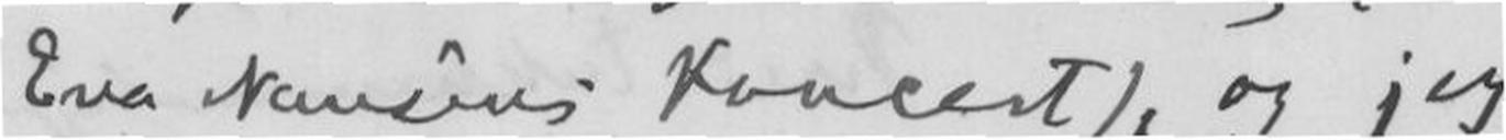Eva Nansens Koncert), og jeg
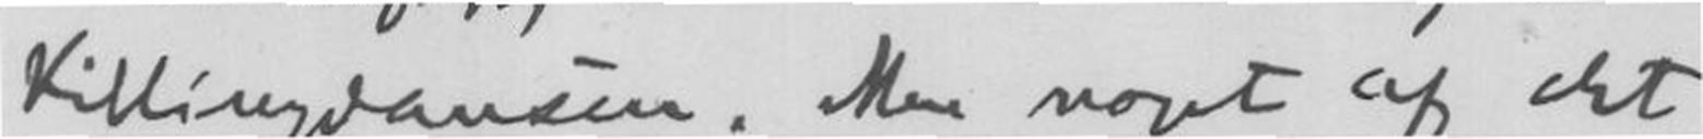Killingdansen. Men noget af det
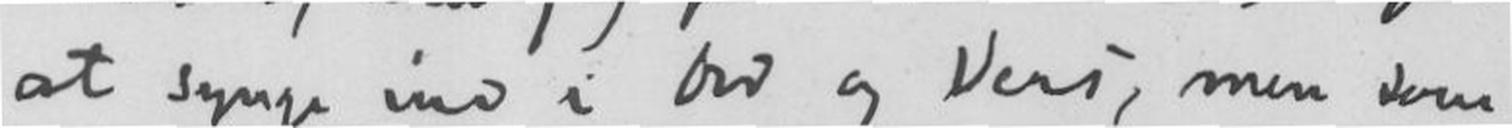at synge ind i Ord og Vers, men som
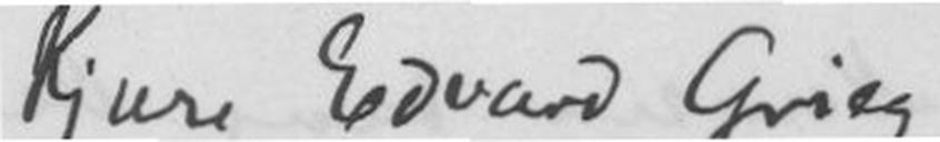Kjære Edvard Grieg.
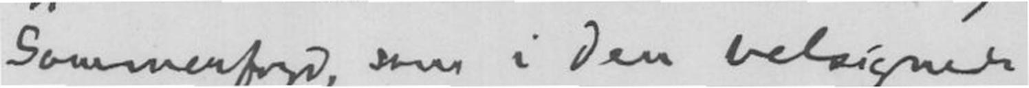Sommerfryd, som i den velsignede
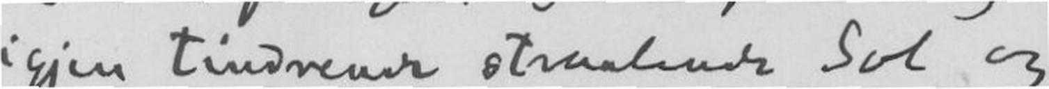igjen tindrende straalende Sol og
